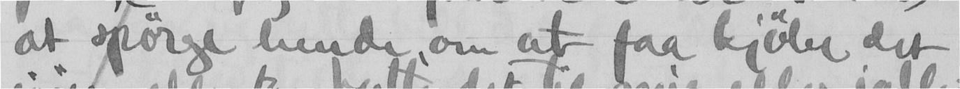at spørge hende, om at faa kjøbe det
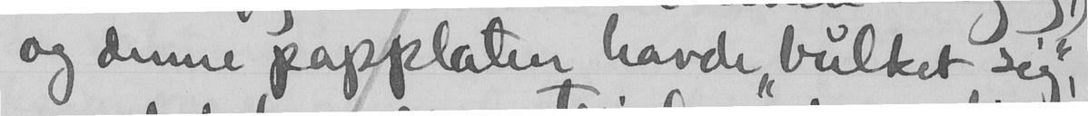og denne papplaten havde "bulket sig",
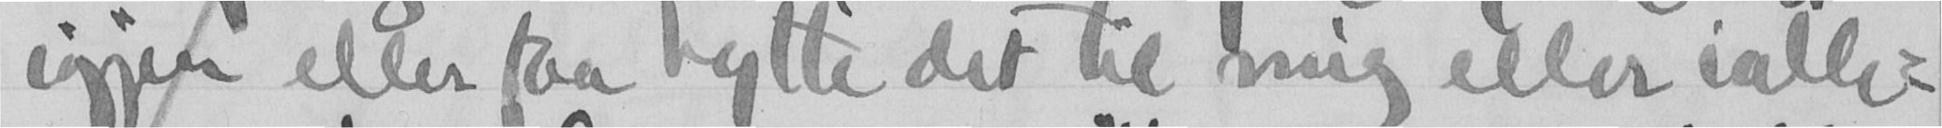igjen eller saa bytte det til mig eller ialle-
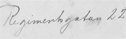Regimentsgatan 22
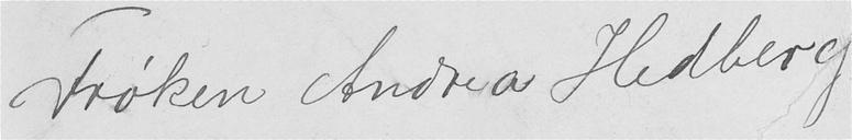Frøken Andrea Hedberg
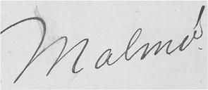Malmø.
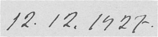12.12.1927.
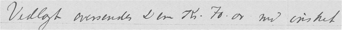Vedlagt oversendes Dem Kr. 70.00 med ønsket
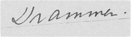Drammen.
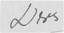Deres
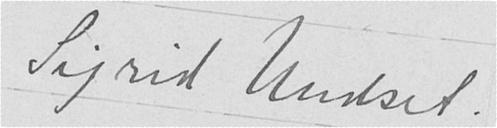Sigrid Undset.
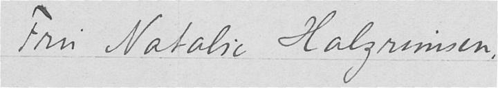Fru Natalie Halgrimsen,
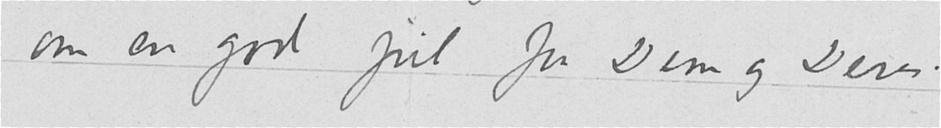om en god jul for Dem og Deres.
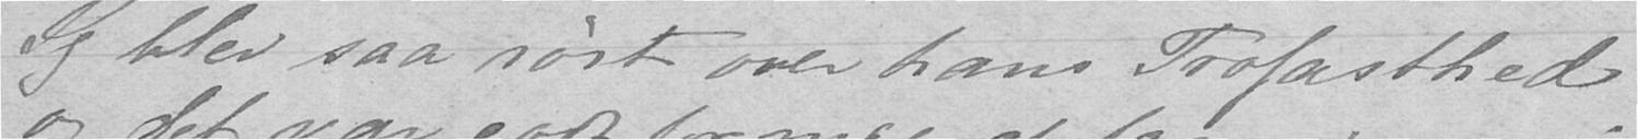Jeg blev saa rørt over hans Trofasthed
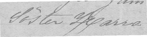Søster Harra
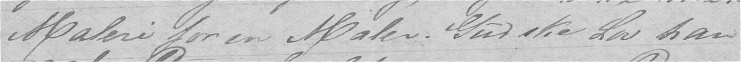Maleri for en Maler. Gud ske Lov han
